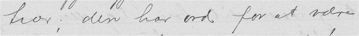trær; den har ord for at være
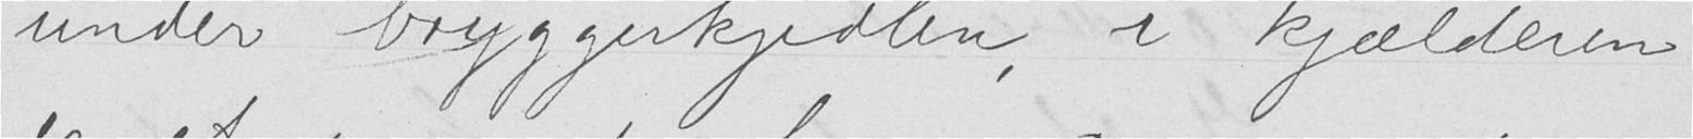under bryggekjedelen, i kjælderen
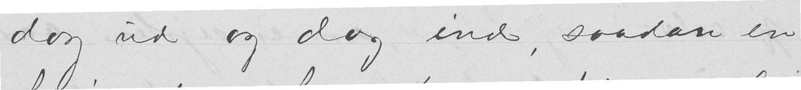dag ud og dag ind, saadan en
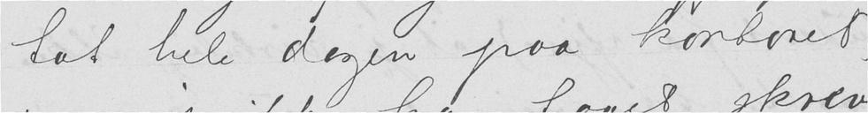tat hele dagen paa kontoret,
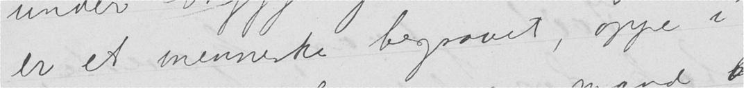er et menneske begravet, oppe i
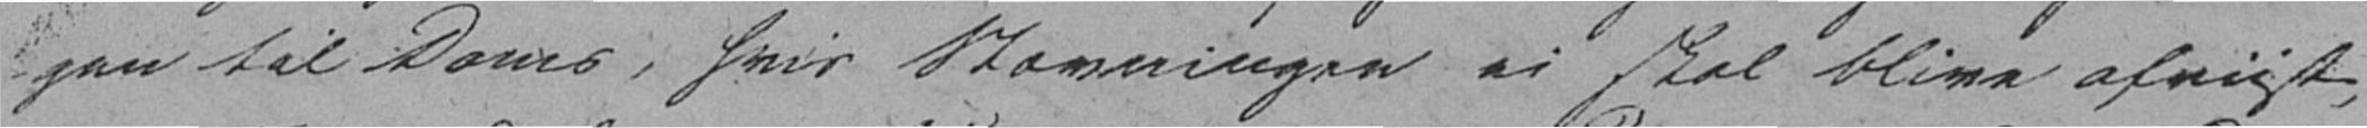gen til Doms, hvis Stævningen ei skal blive afvist,
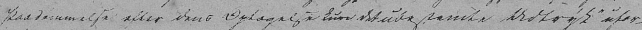Paadømmelse efter dens Optagelse kun det ubestemte Udtryk "ufor-
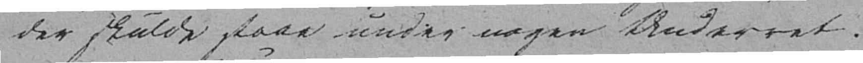der skulde staae under nogen Underret.
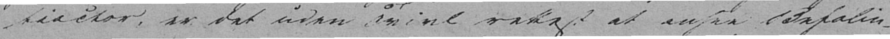tiactor, er det uden Tvivl rettest at ansee Befalin-
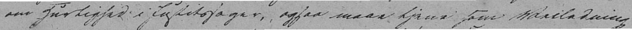om Hurtighed i Justitssager, ogsaa maae tjene som Veiledning
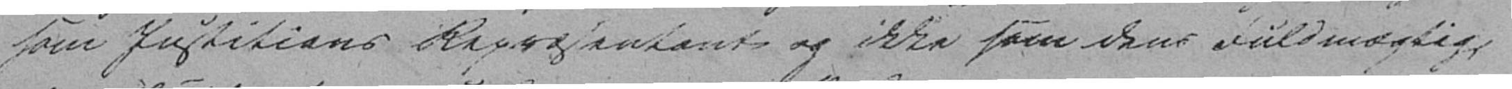som Justitsens Representant og ikke som dens Fuldmægtig,
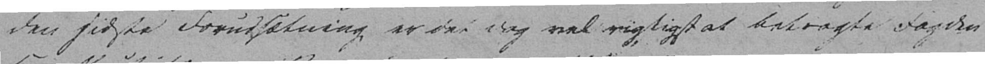den sidste Forudsætning er der dog vel rigtigt at betragte Fogden
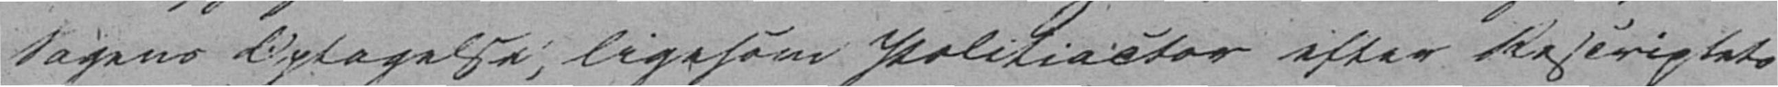Sagens Optagelse, ligesom Politiactor efter Rescriptets
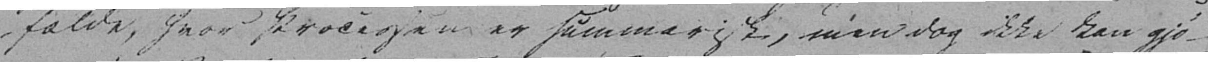fælde, hvor Processen er summarisk, men dog ikke kan gjø-
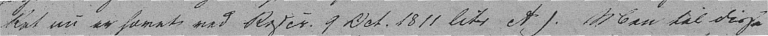Ret nu er hævet ved Rescr. 9 Oct. 1811 litr A). Men til disse
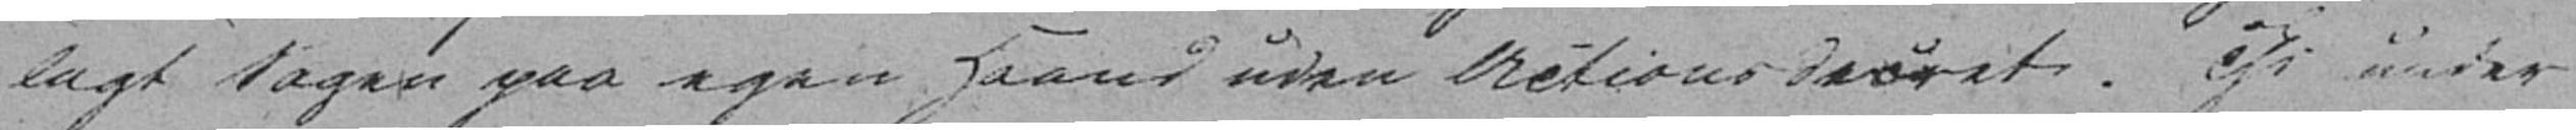lagt Sagen paa egen Haand uden Actionsdecret. Thi under
